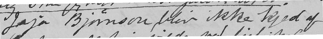Jaja Bjørnson, bliv ikke kjed af
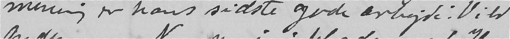mening er hans sidste gode arbejde: Vild
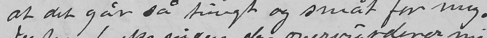at det går så tungt og småt for mig.
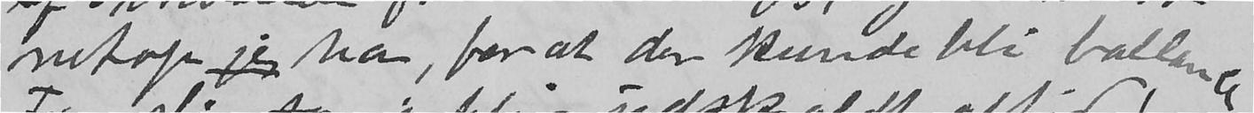netop jeg ha, for at der kunde bli ballance.
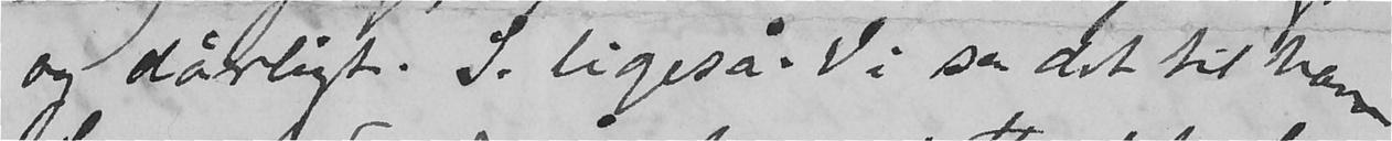og dårligt. S. ligeså. Vi sa det til ham.
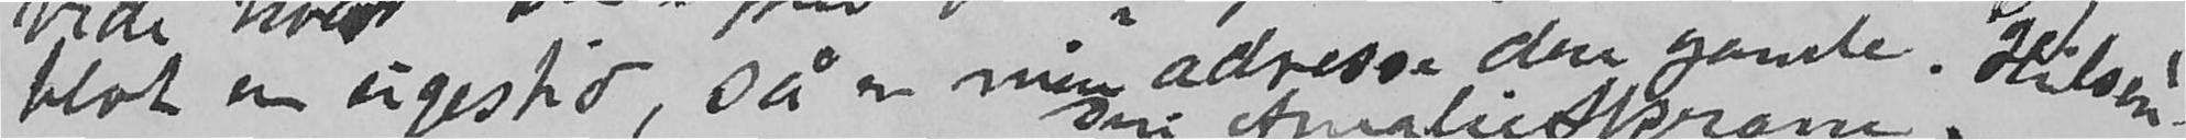blot en ugestid, så er min adresse den gamle. Hilsen
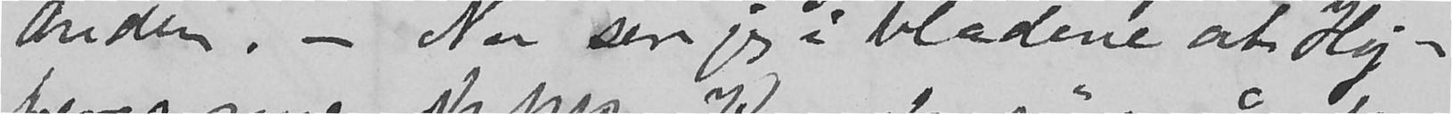anden. - Nu ser jeg i bladene at Hej-
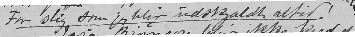For slig som jeg blir udskjældt altid!
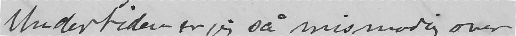Undertiden er jeg så mismodig over
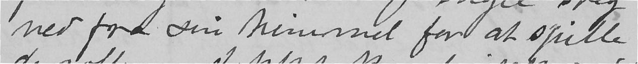ned fra sin himmel for at spille
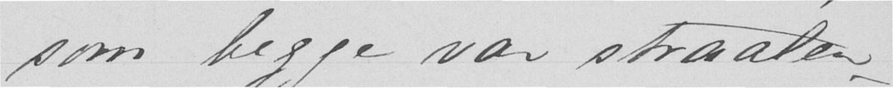som begge var straalen-
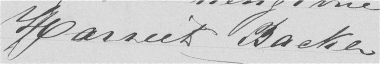Harriet Backer
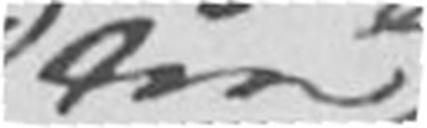saa
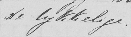de lykkelige.
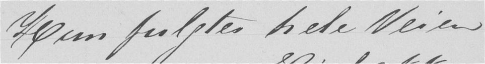Hun fulgtes hele Veien
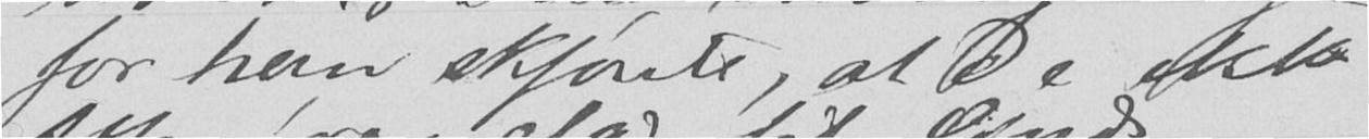for han skjønte, at De ikke
